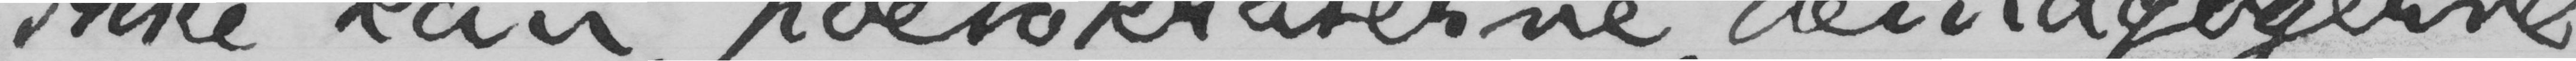ikke kan, poetokraterne, demagogerne,
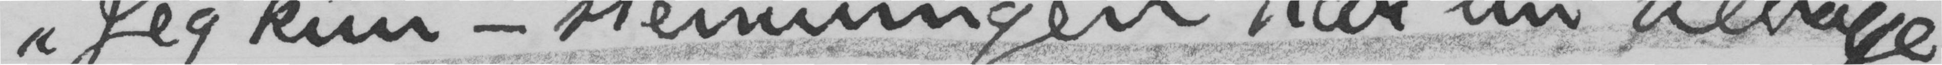«Jeg kun – stemningen har nu tilbage,
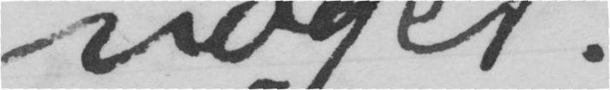noget.
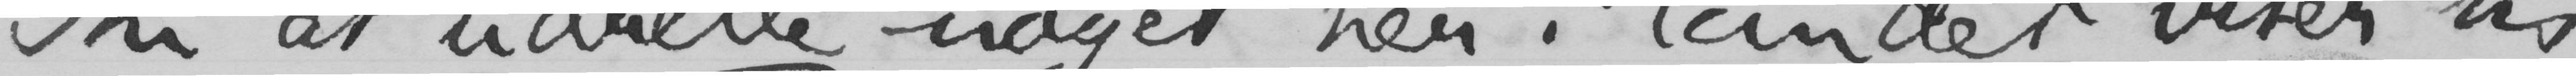Thi at udrette noget her i landet viser sig
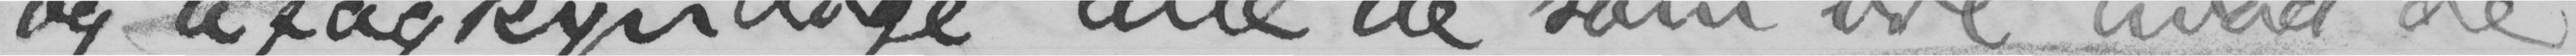og ufagkyndige, alle de, som vil, hvad de
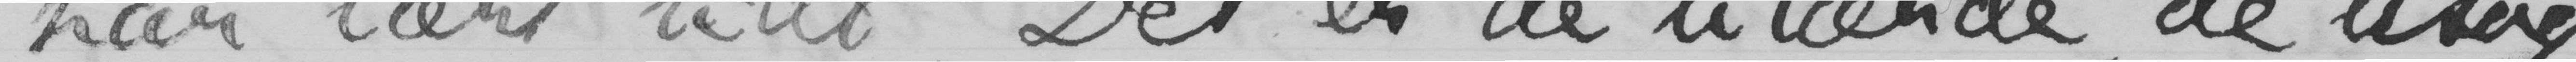har lært lidt. Det er de ulærde, de usag-
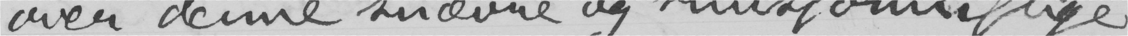over denne snævre og snusfornuftige
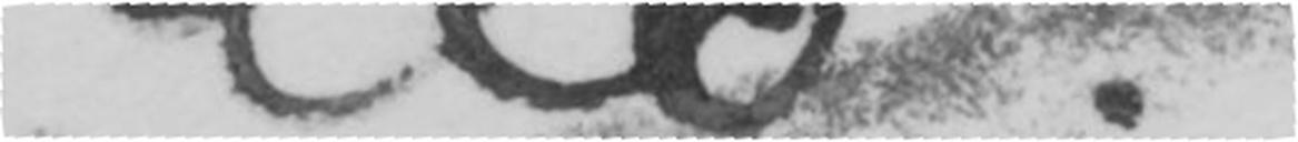illo.
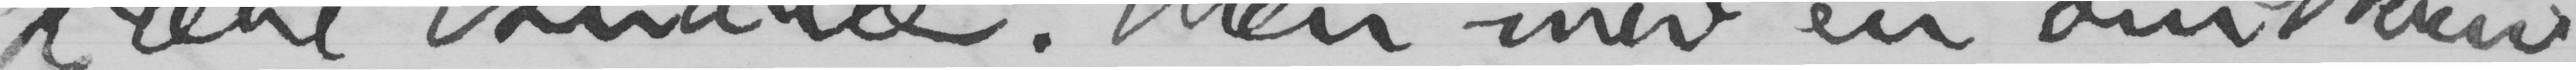kjære Andræ. Men med en omskriv-
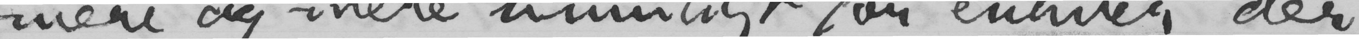mere og mere umuligt for enhver, der
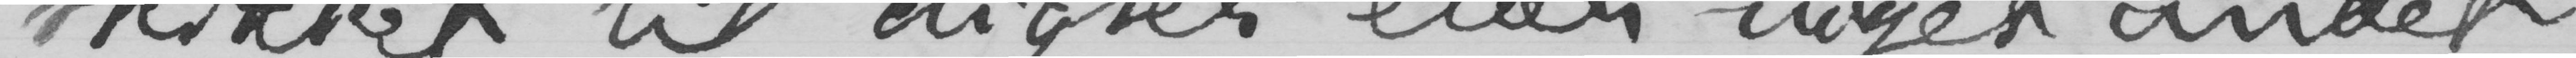skikket til digter eller noget andet
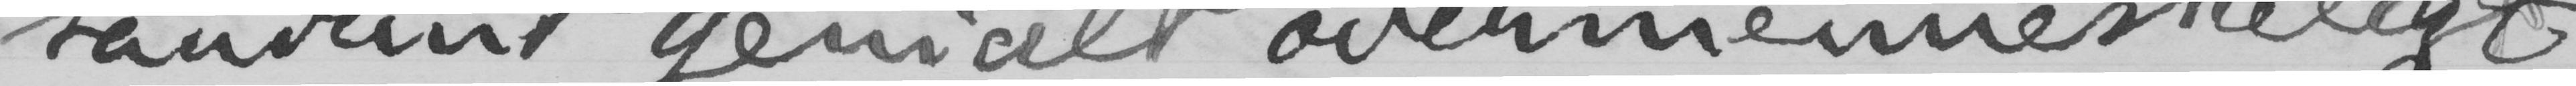saadant genialt overmenneskeligt
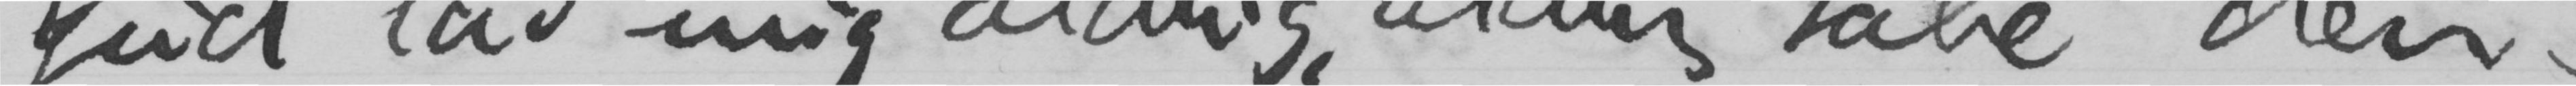Gud lad mig aldrig, aldrig tabe den» -
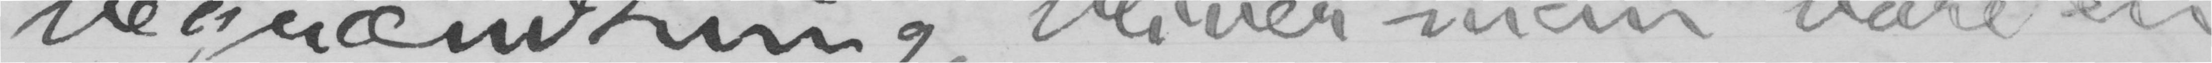begrændsning, bliver man bare en
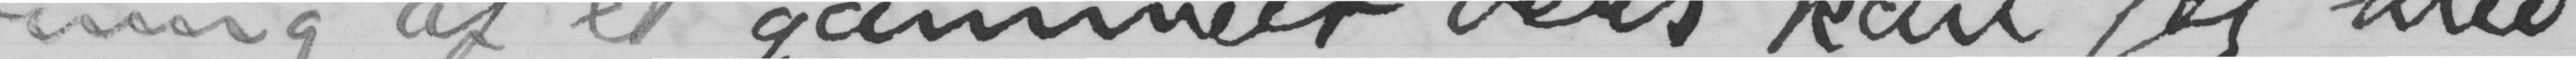ning af et gammelt vers kan jeg med
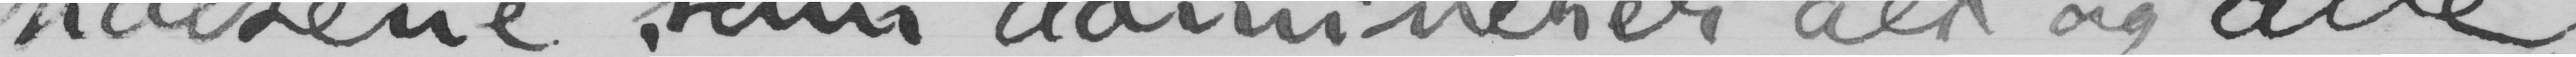halserne, som dominerer alt og alle
